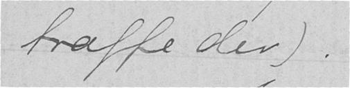træffe der).
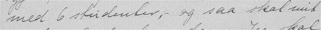med 6 studenter, - og saa skal mit
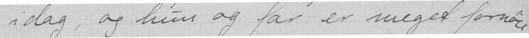i dag, og hun og far er meget fornøie-
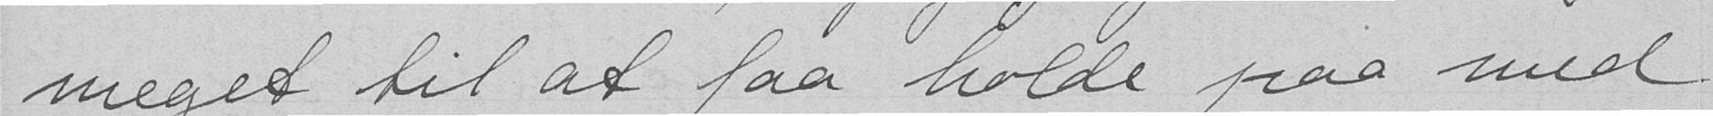meget til at faa holde paa med
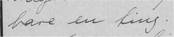bare en ting.
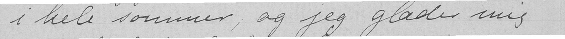i hele sommer, og jeg glæder mig
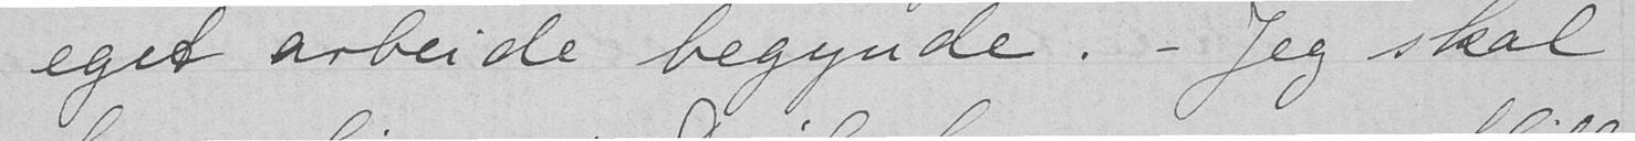eget arbeide begynde. - Jeg skal
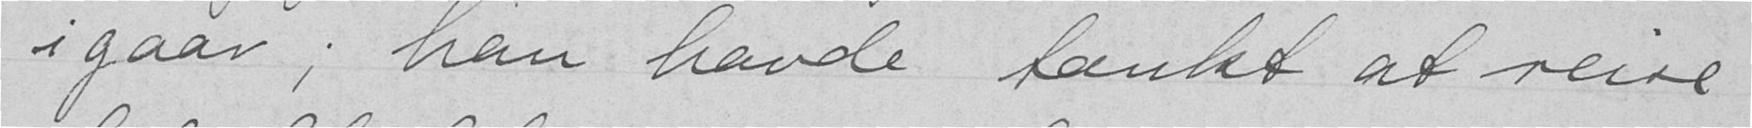i gaar; han havde tænkt at reise
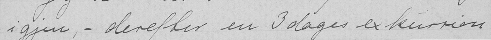igjen, - derefter en 3 dages exkursion
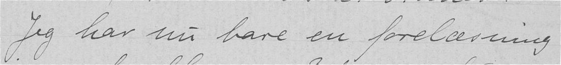- Jeg har nu bare en forelæsning
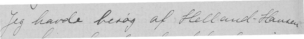Jeg havde besøg af Helland-Hansen
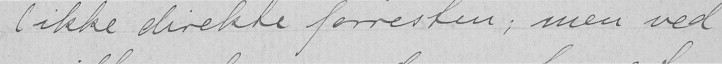(ikke direkte forresten; men ved
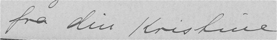fra din Kristine.
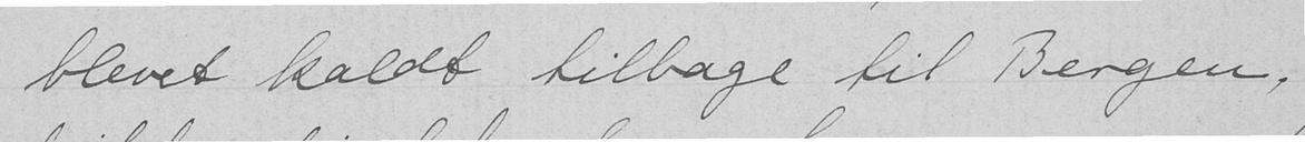blevet kaldt tilbage til Bergen,
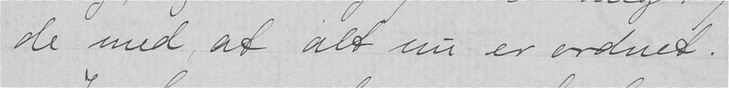de med, at alt nu er ordnet.
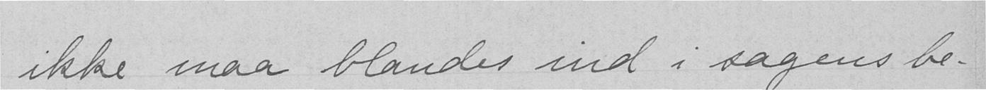ikke maa blandes ind i sagens be-
